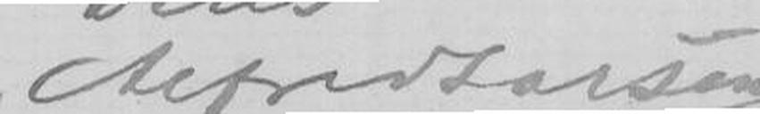Alfred Larsen
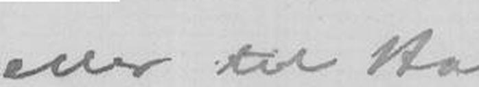eller til Hals.
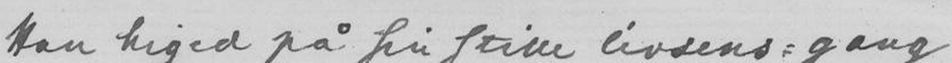Han higed på sin stille livsens=gang
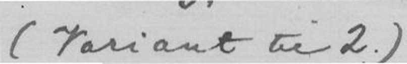(Variant til 2.)
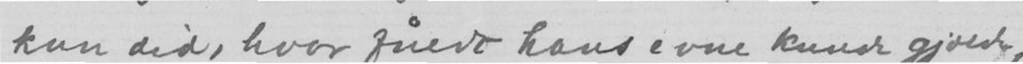kun did, hvor findt hans evne kunde gjælde,
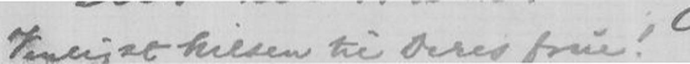Venligst hilsen til Deres frue !
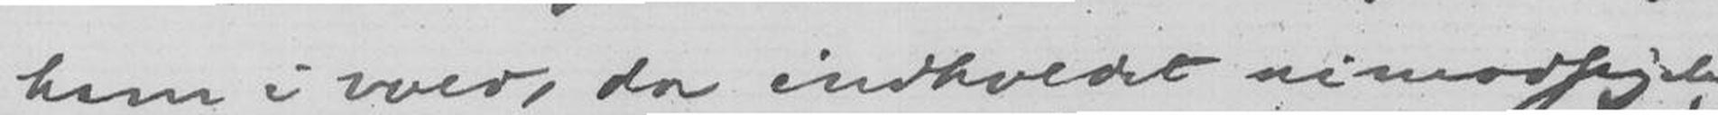ham i voer, da indholdet uimodsigetig
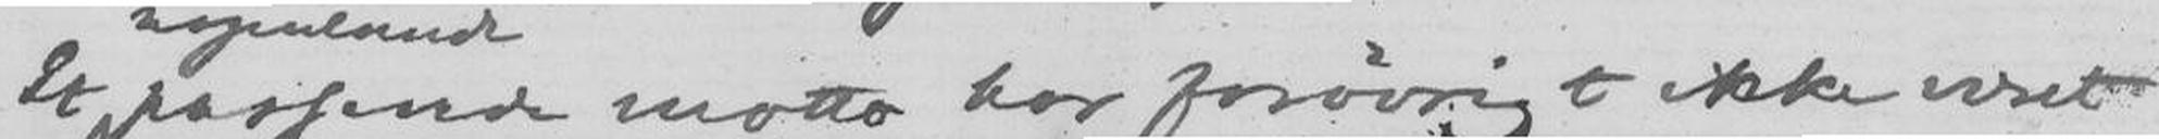Et passende motto har forøvrigt ikke været
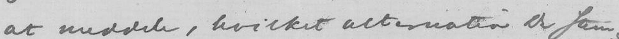at meddele, hvilket alternativ De stem-
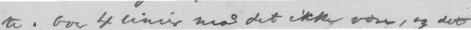te. Over 4 linier må det ikke være, og det
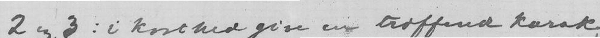2 og 3: i korthed give en træffende karak-
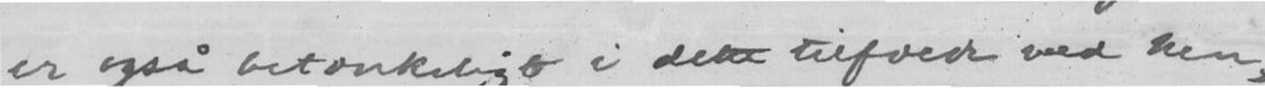er også betænkeligt i dette tilfælde ved hen-
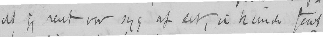at jeg rent var syg af det, vi kunde faat
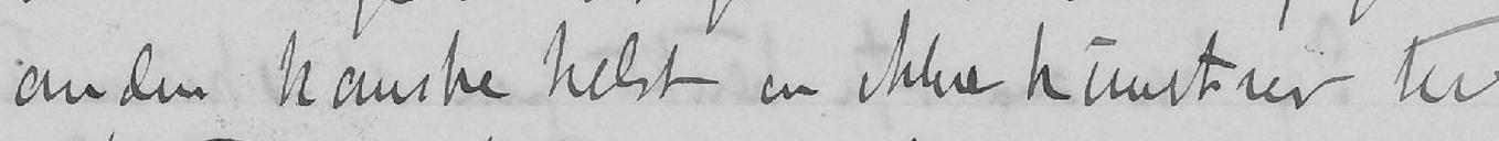anden kanske helst en ikke kunstner til
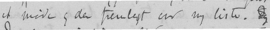et møde og der fremlagt en ny liste. Dg
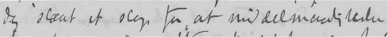dog slaaet et slag for, at middelmaadigheden
